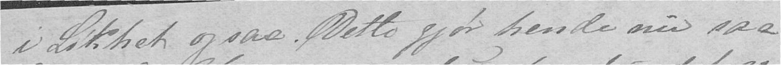i Likhet ogsaa. Dette gjør hende nu saa
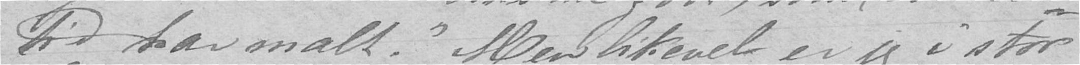tid har malt." Men likevel er jeg i stor
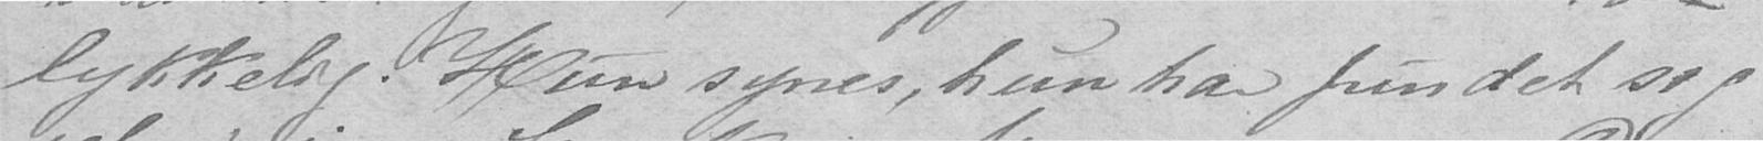lykkelig. Hun synes, hun har fundet sig
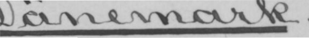Dänemark.
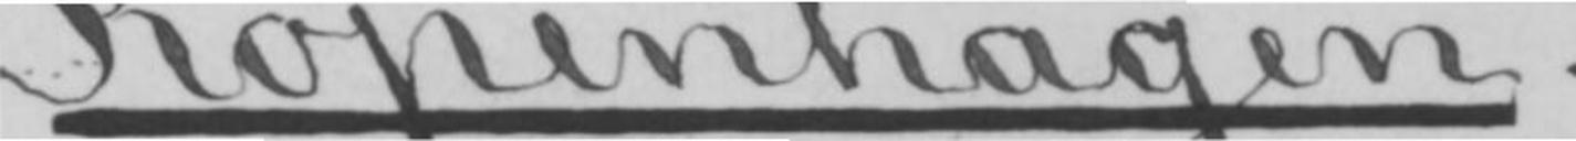Kopenhagen.
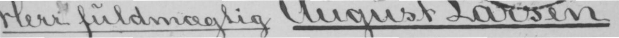Herr fuldmægtig August Larsen.
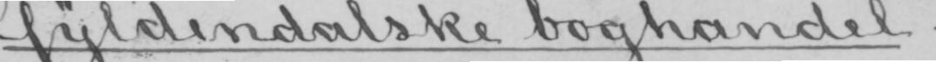Gyldendalske boghandel.
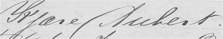Kjære Aubert.
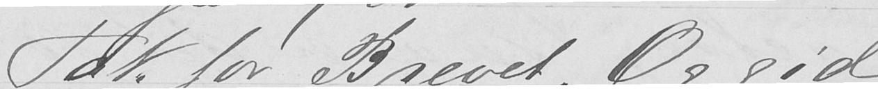Tak for Brevet. Og gid
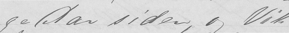ge Aar siden og Vik
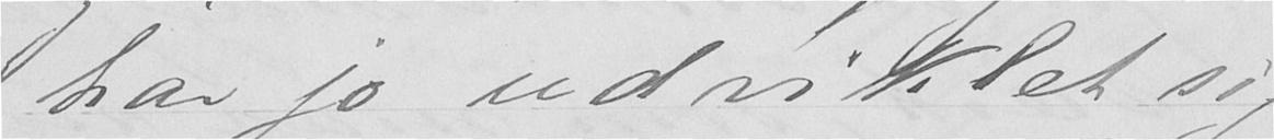har jo udviklet sig
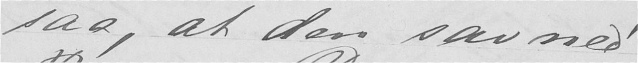saa, at den savned
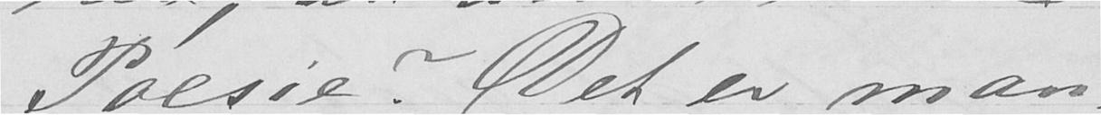Poesie? Det er man-
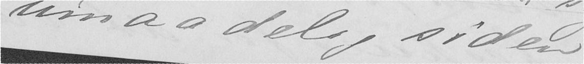umaadelig siden
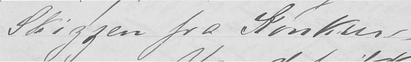Skizzen fra Konkur-
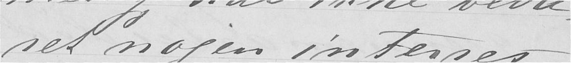ret nogen interes-
